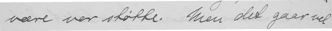være vor støtte. Men det gaar vel
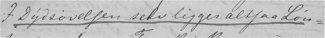I Dydsøvelsen selv ligger altsaa Løn-
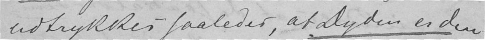udtrykkes saaledes, at Dyden er den
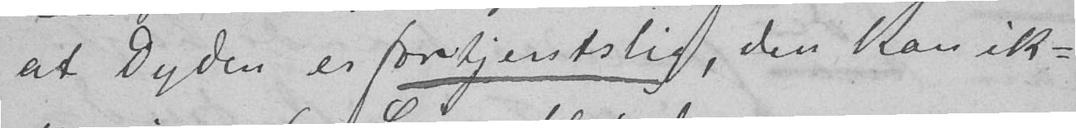at Dyden er fortjenstlig, den kan ik-
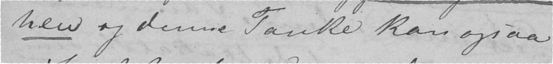nen og denne Tanke kan ogsaa
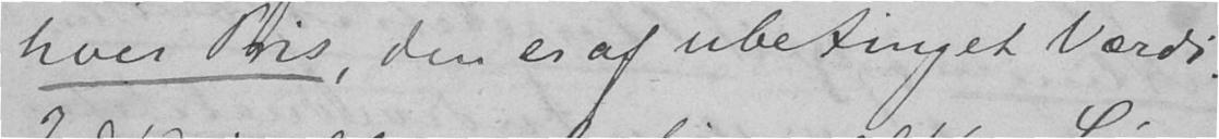hvis Pris, den er af ubetinget Værdi.
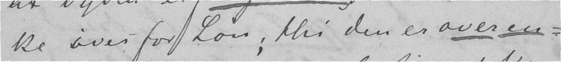ke øves for Lov; thi den er over en-
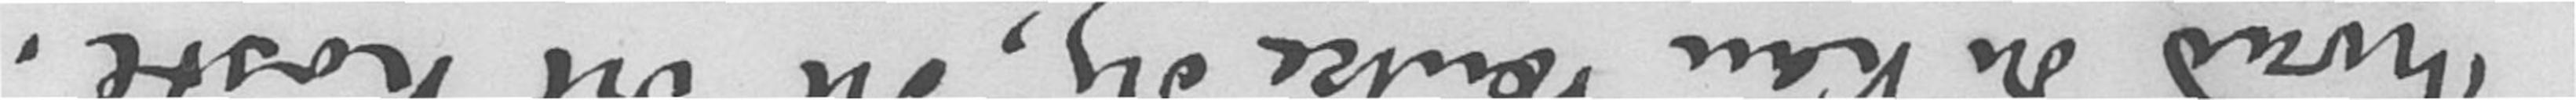hvad du kan tænke dig, det vil koste.
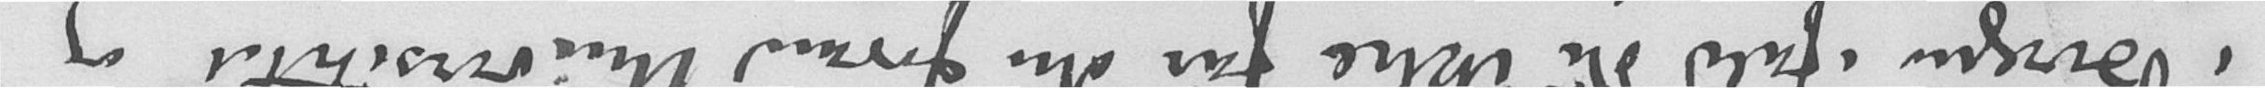i Bergen ifald du ikke får den foran universitet og
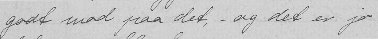godt mod paa det, - og det er jo
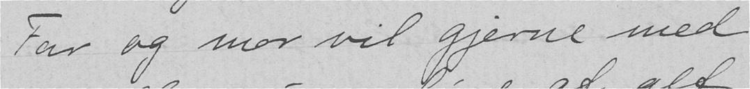Far og mor vil gjerne med
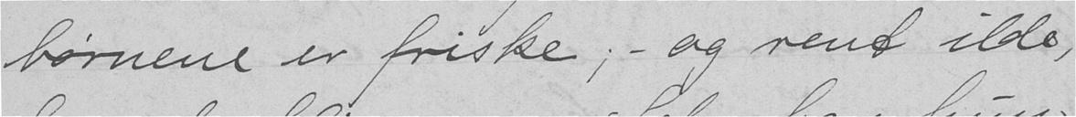børnene er friske; - og rent ilde,
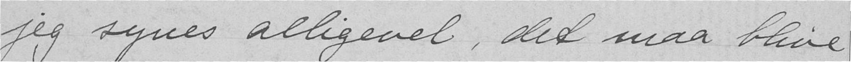jeg synes alligevel, det maa blive
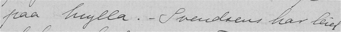paa Mylla. - Svendsens har leiet
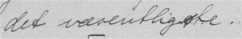det væsentligste.
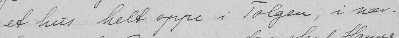et hus helt oppe i Tolgen, i nær-
